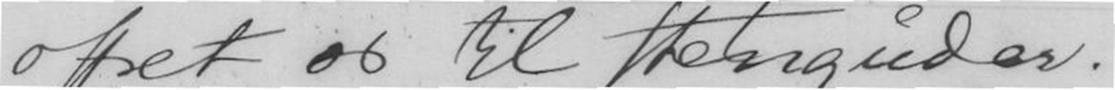offret os til stenguder.
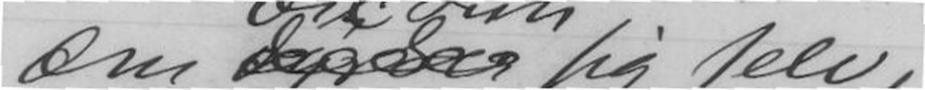den vil kun sig selv,
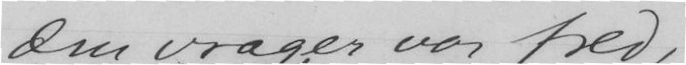den vrager vor fred,
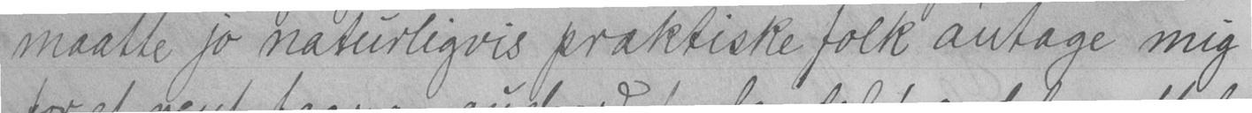maatte jo naturligvis praktiske folk antage mig
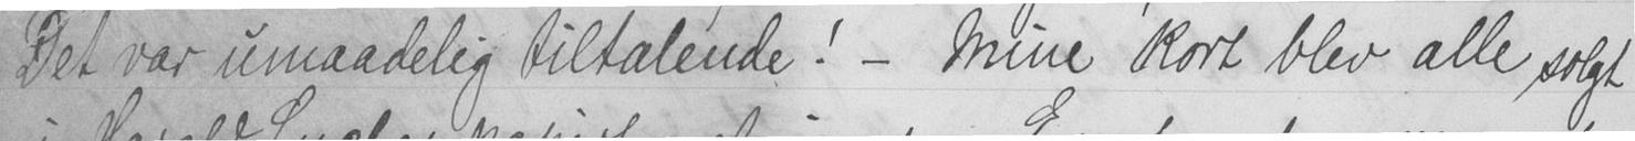Det var umaadelig tiltalende! - mine kort blev alle solgt
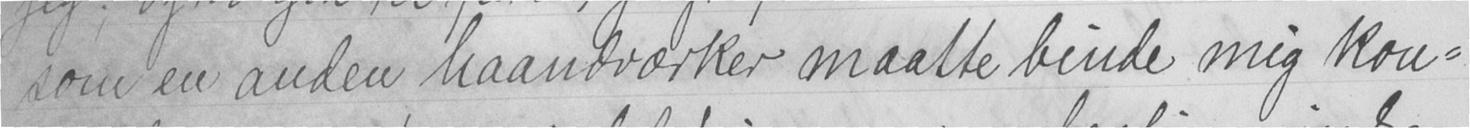som en anden haandværker maatte binde mig kon-
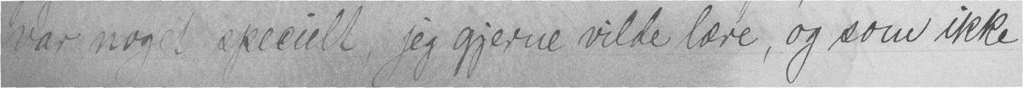var noget specielt, jeg gjerne vilde lære, og som ikke
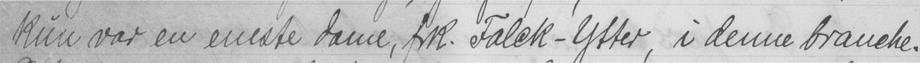kun var en eneste dame, frk. Falck-Ytter, i denne branche.
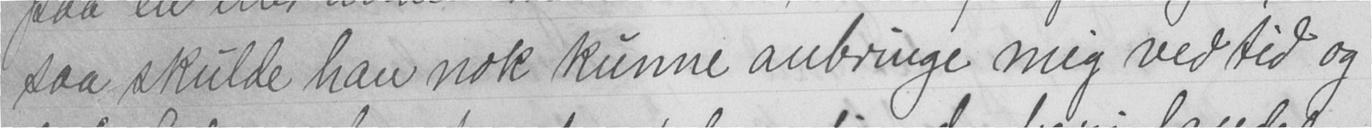saa skulde han nok kunne anbringe mig ved tid og
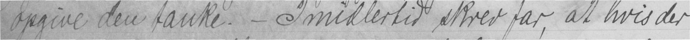opgive den tanke. - Imidlertid skrev far, at hvis der
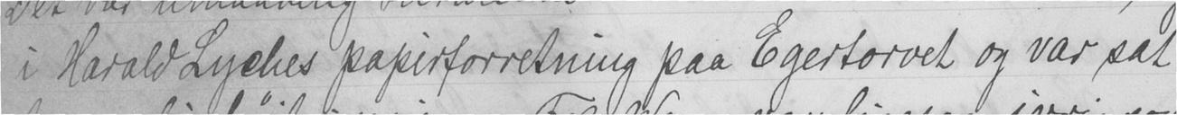i Harald Lyches papirforretning paa Egertorvet og var sat
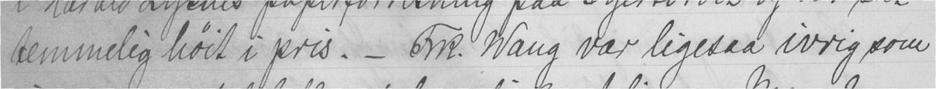temmelig høit i pris. - Frk. Wang var ligesaa ivrig som
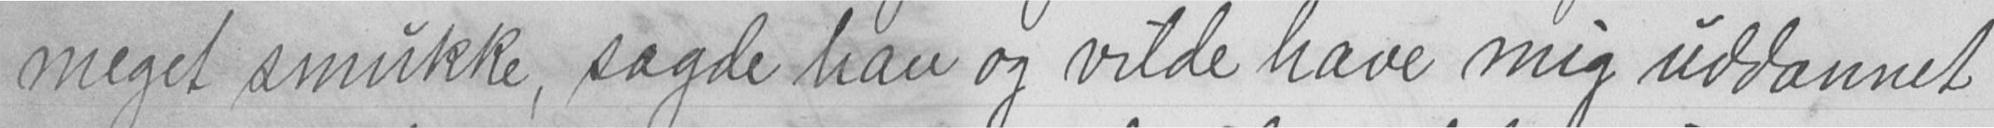meget smukke, sagde han og vilde have mig uddannet
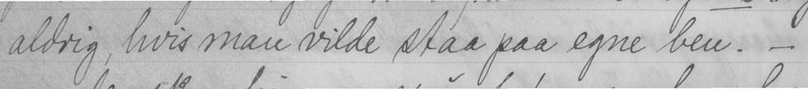aldrig, hvis man vilde staa paa egne ben. -
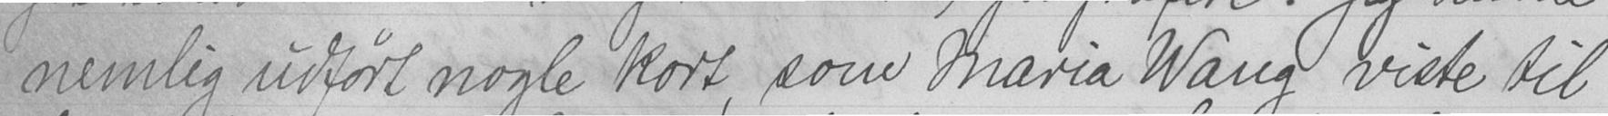nemlig udført nogle kort, som Maria Wang viste til
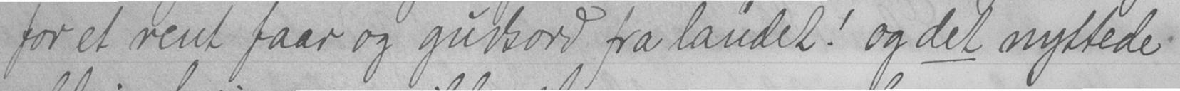for et rent faar og gudsord fra landet! og det nyttede
